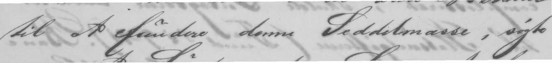til at fundere denne Seddelmasse, søgte
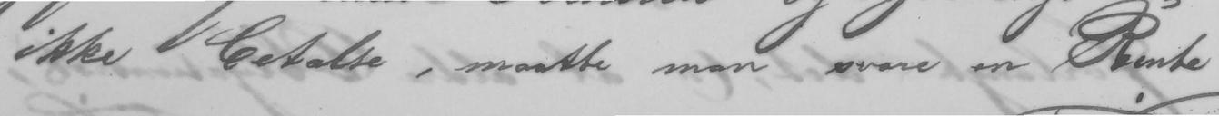ikke betalte, måtte man svare en Rente
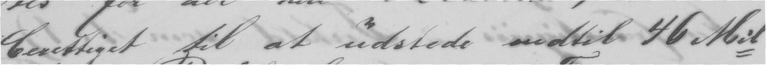berettiget til at udstede indtil 46 Mil-
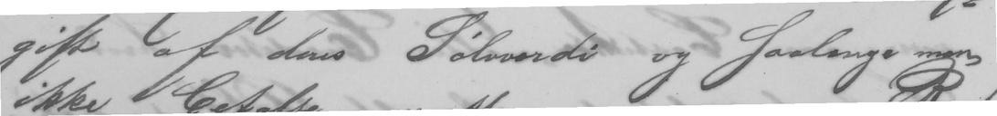gift af dens Sølvværdi og Saalenge man
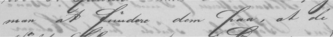man at fundere dem paa, at de
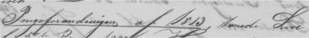Pengeforandringen af 1813, tvende Love
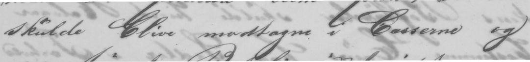skulde blive modtagne i Casserne og
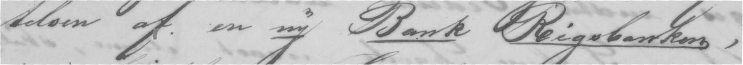telsen af en ny Bank Rigsbanken,
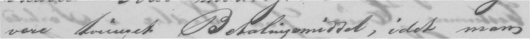vare tvunget Betalingsmiddel, idet man
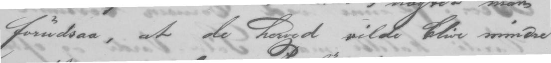forudsaa, at de herved vilde blive mindre
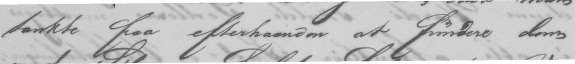tenkte paa efterhaanden at fundere dem
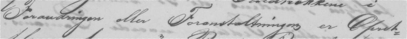Forandringen eller Foranstaltningen er Opret-
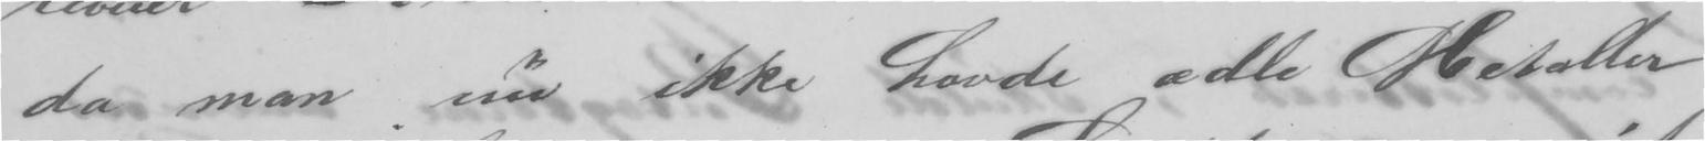da man nu ikke havde ædle Metaller
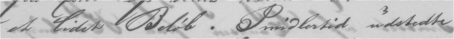et lidet Beløb. Imidlertid udstedte
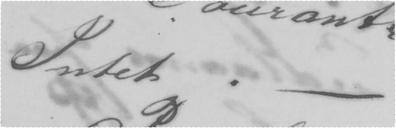Intet. -
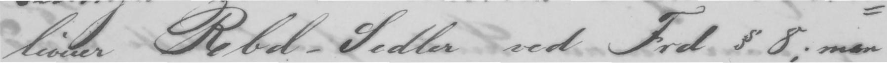lioner Rbd-Sedler ved Frd §.8; men
